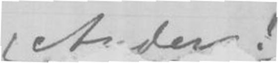Anders!
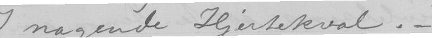I nagende Hjertekval.-
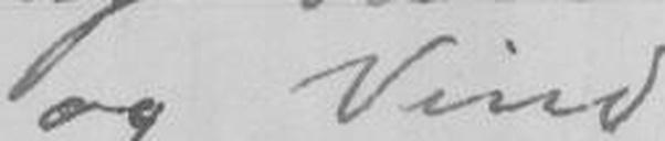og Vind,
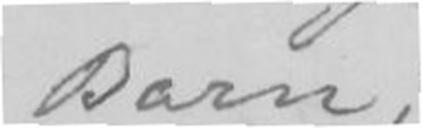Barn,-
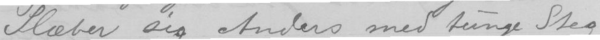Slæber sig Anders med tunge Steg
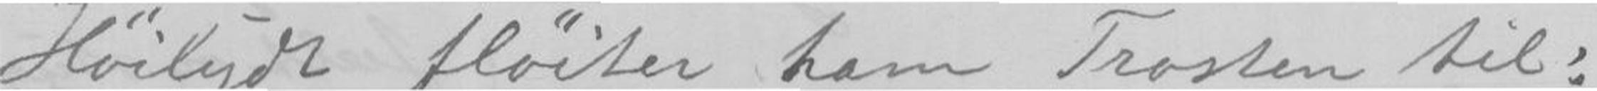Høilydt fløiter ham trosten til:
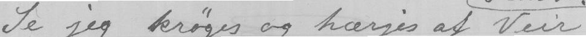"Se jeg krøges og hærjes af Veir
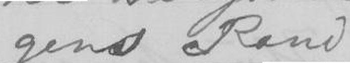gens Rand
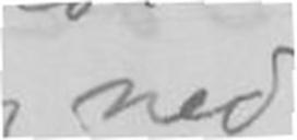ned
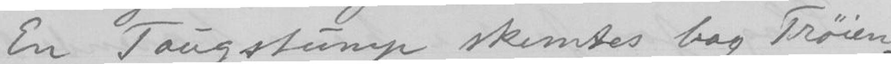En Taugstump skimtes bag Trøien.
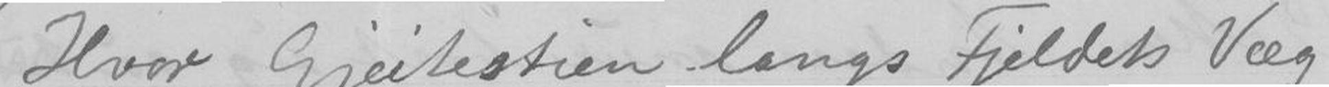Hvor Gjeitestien langs Fjeldets Væg
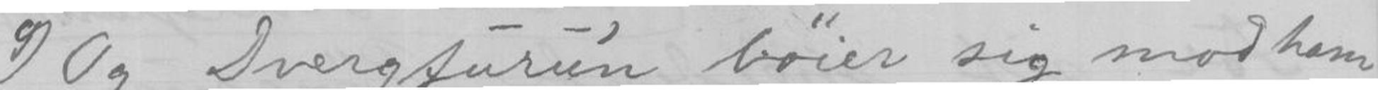Og Dvergfuru'n bøier sig mod ham
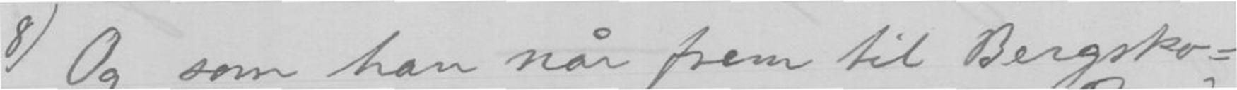Og som han når frem til Bergsko-
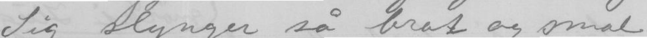Sig slynger så brat og smal
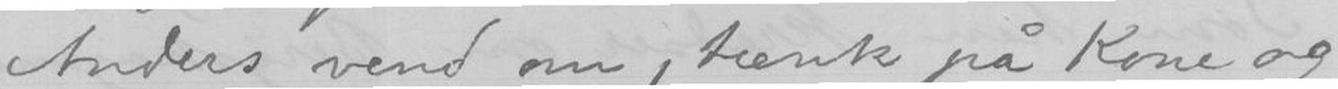"Anders vend om, tænk på Kone og-
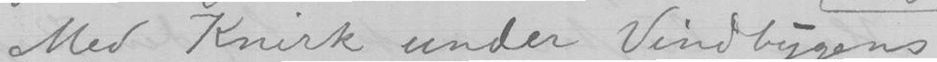Med Knirk under Vindbygens
